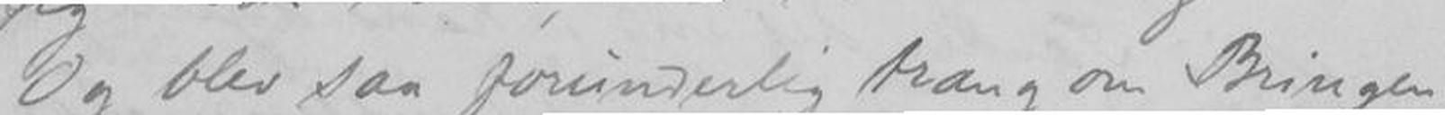Og blev saa forunderlig trang om Bringen,
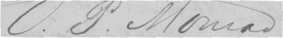O. P. Monrad
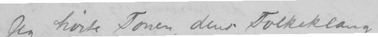Jeg hørte Tonen, dens Folkeklang
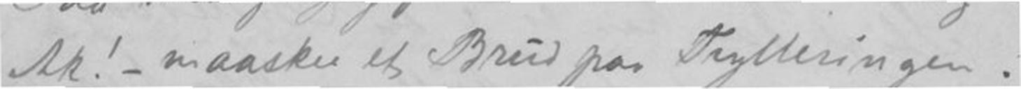Ak! - maaske et Brud paa Trylleringen!
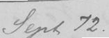Sept. 72.
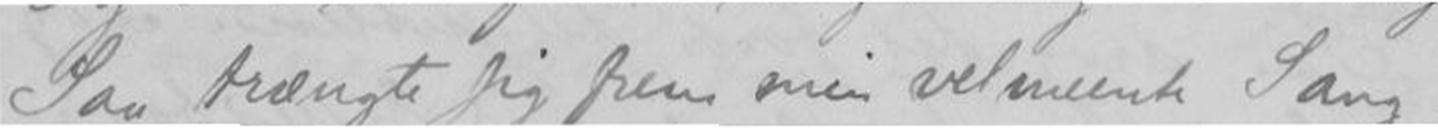Saa trængte sig frem min velmente Sang -
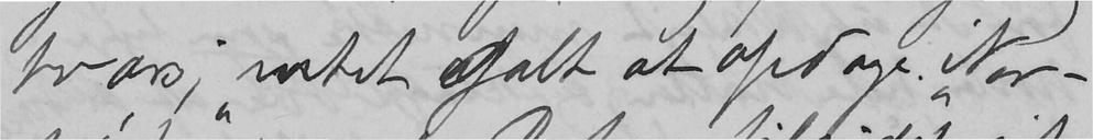tværs; intet galt at opdage. "Ner-
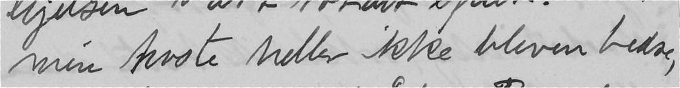min hoste heller ikke bleven bedre,
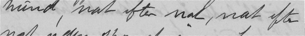hund, nat efter nat, nat efter
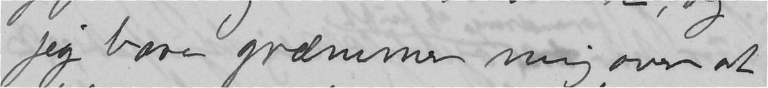jeg bare græmmer mig over at
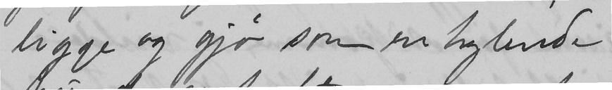ligge og gjø som en hylende
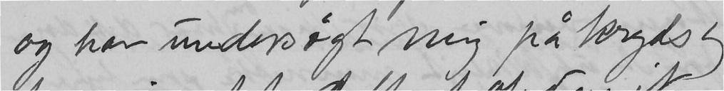og har undersøgt mig på krydst og
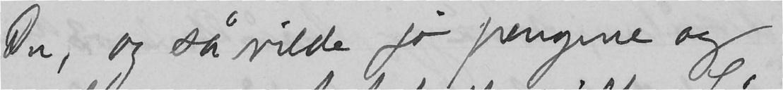Du, og så vilde jo pengene og
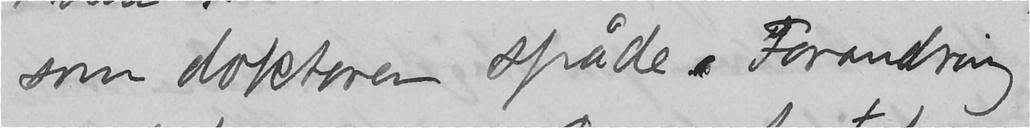som doktoren spåde. Forandring
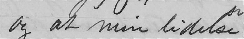og at min lidelse
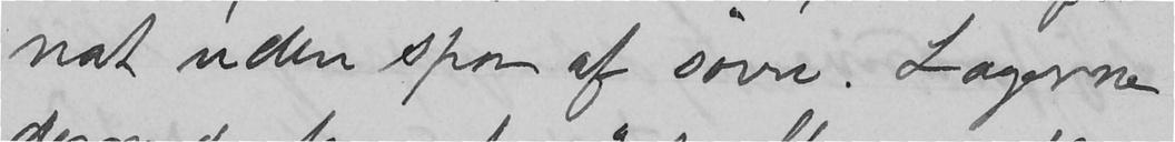nat uden spor af søvn. Lagerne
